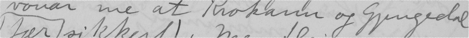vonar me at Krokann og Gjengedal
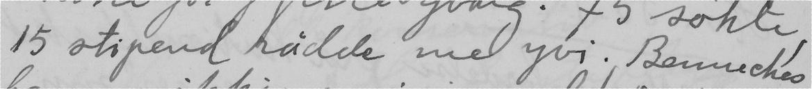15 stipend nådde me yvi. Benneches
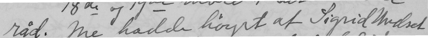råd. Me hadde höyrt af Sigrid Undset
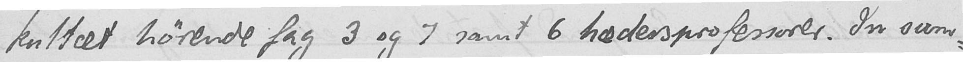kultet hørende fag 3 og 7 samt 6 hædersprofessorer. In sum-
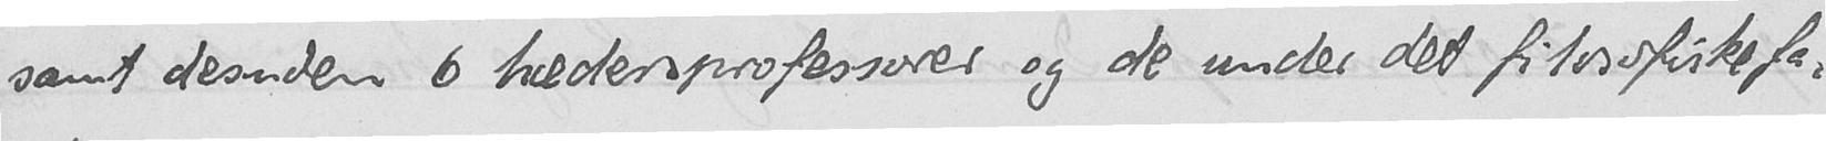samt desuden 6 hædersprofessorer og de under det filosofiske fa-
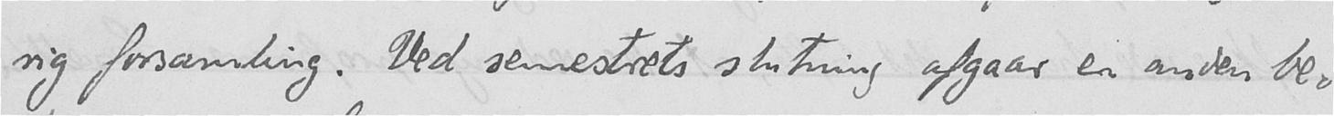rig forsamling. Ved semestrets slutning afgaar en anden be-
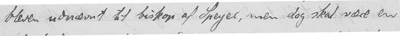bleven udnævnt til biskop af Speyer, men dog skal være en
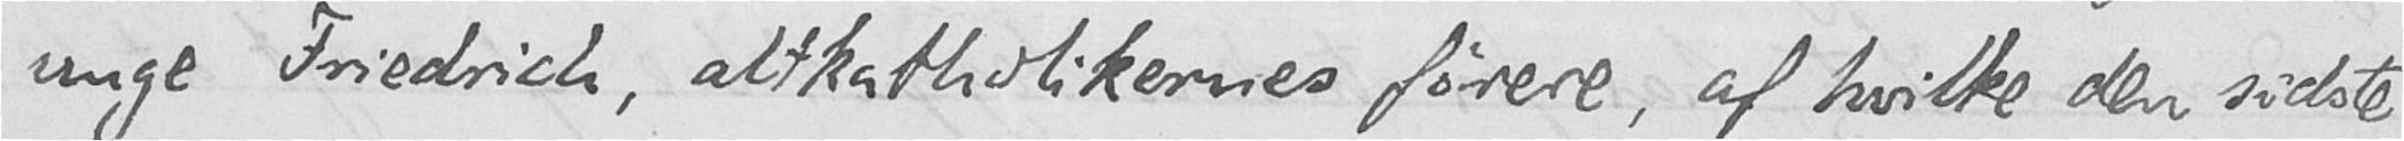unge Friedrich, altkatholikernes førere, af hvilke den sidste
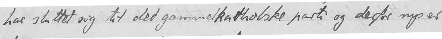har sluttet sig til det gammelkatholske parti og derfor nys er
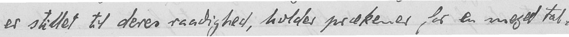er stillet til deres raadighed, holder prækener for en meget tal-
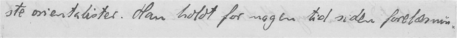ste orientalister. Han holdt for nogen tid siden forelæsnin-
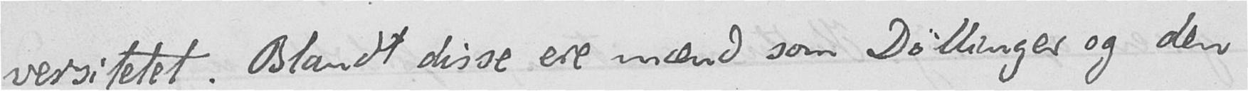versitetet. Blandt disse ere mænd som Döllinger og den
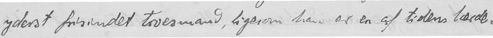yderst frisindet troesmand, ligesom han er en af tidens lærde-
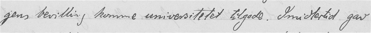gens bevilling komme universitetet tilgode. Imidlertid gav
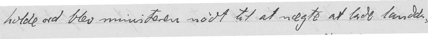holde ord blev ministeren nødt til at nægte at lade landda-
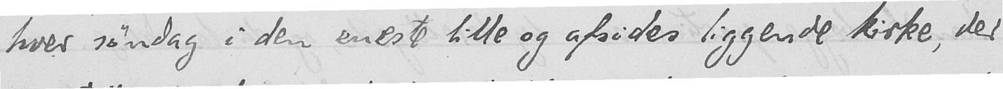hver søndag i den eneste lille og afsides liggende kirke, der
